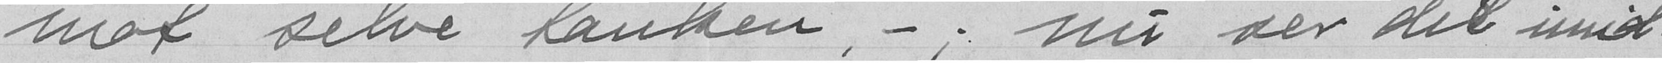mot selve tanken -; nu set det imid-
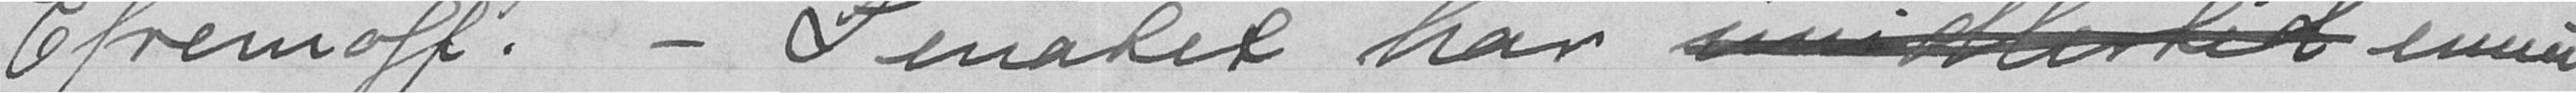Efremoff. - Senatet har imidlertid ennu
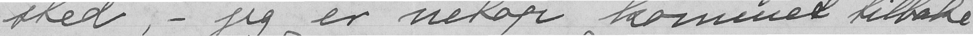sted, - jeg er netop kommet tilbake
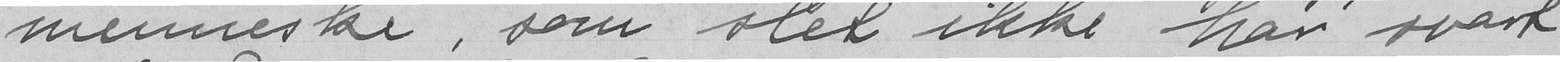menneske, som slet ikke har svart
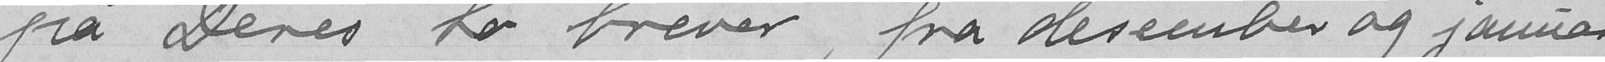på Deres to brever, fra desember og januar.
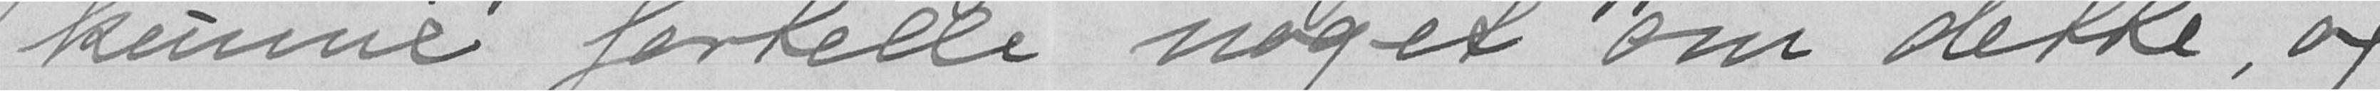kunne fortelle noget om dette, og
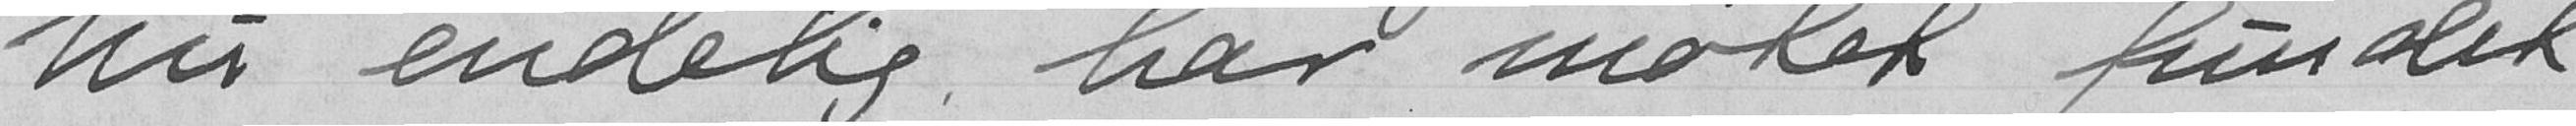Nu endelig har møtet fundet
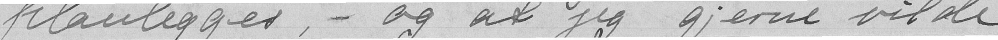planlegges, - og at jeg gjerne vilde
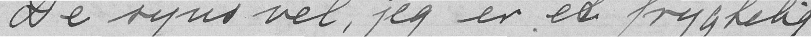De syns vel, jeg er et frygtelig
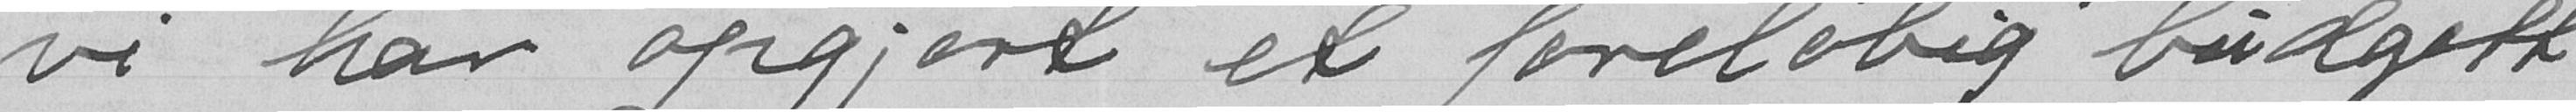vi har opgjort et foreløbig budgett
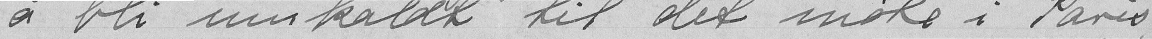å bli innkaldt til det møte i Paris,
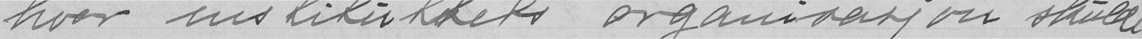hvor instituttets organisasjon skulle
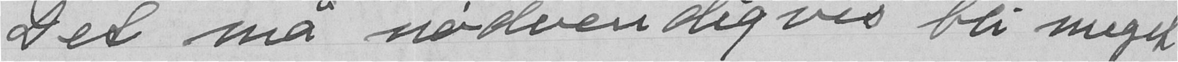Det må nødvendigvis bli meget
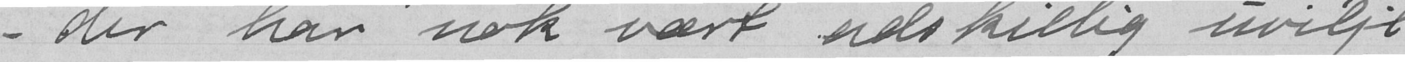- der har nok vært adskillig uvilje
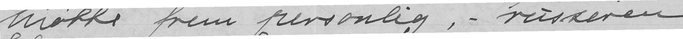møtte frem personlig, - russeren
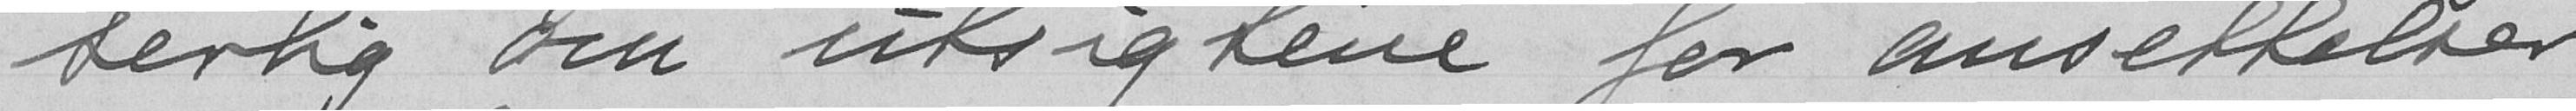dertil om utsigtene for ansettelser.
